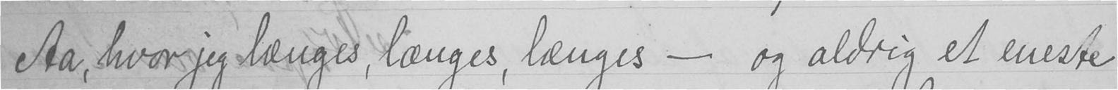Aa, hvor jeg længes, længes, længes - og aldrig et eneste
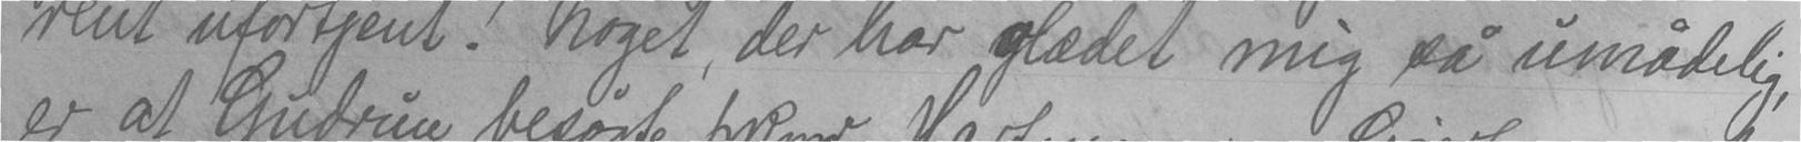rent ufortjent! Noget, der har glædet mig så umådelig,
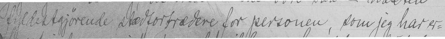fyldestgjørende stædfortrædene for personen, som eg har er-
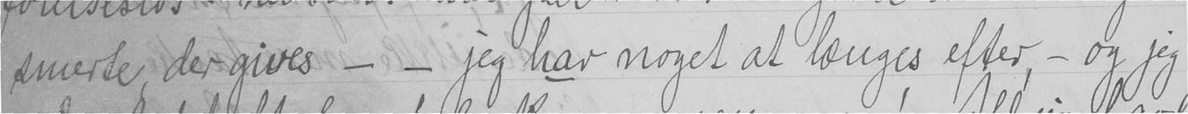smerte, der gives - - jeg har noget at længes efter, - og jeg
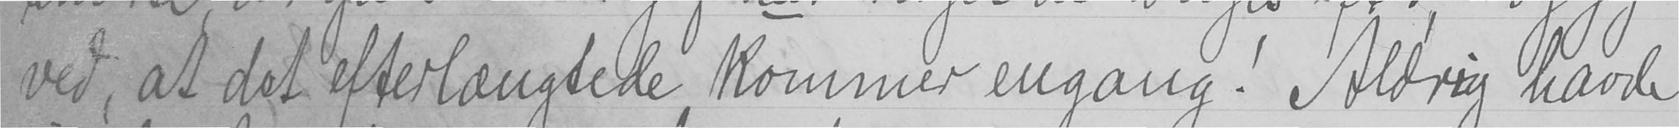ved, at det efterlængtede kommer engang! Aldrig havde
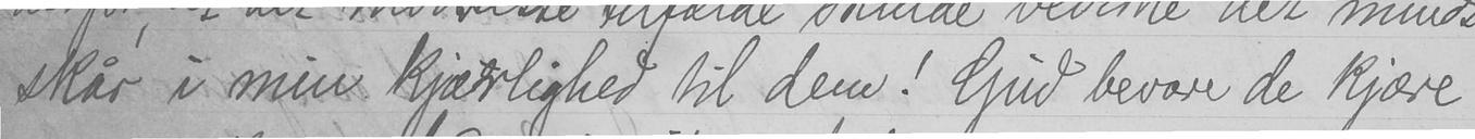skår i min kjærlighed til dem! Gud bevare de kjære
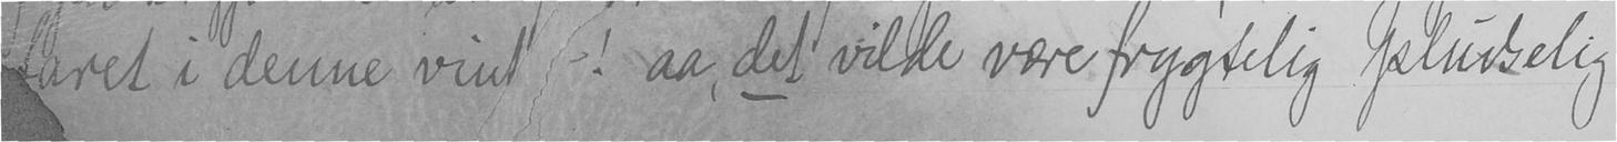faret i denne vinter! aa, det vilde være frygtelig plutselig
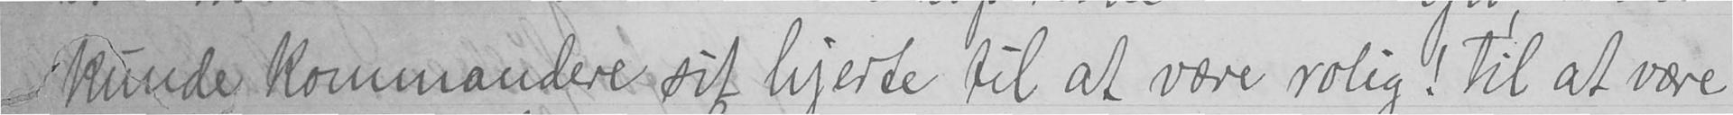kunde kommandere sit hjerte til at være rolig! Til at være
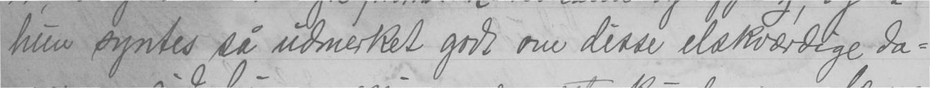hun syntes så udmærket godt om disse elskværdige da-
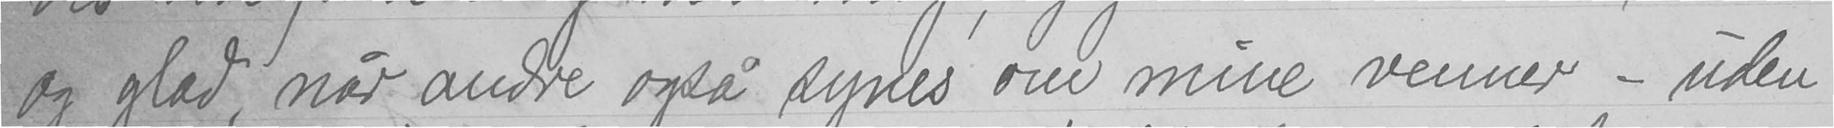og glad, når andre også synes om mine venner - uden
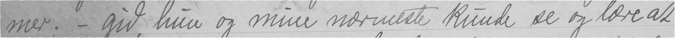mer; - gid, hun og mine nærmeste kunde se og lære at
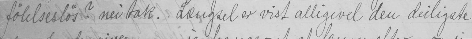følelsesløs? nei tak. Længsel er vist alligevel den deiligste
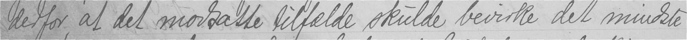derfor, at det modsatte tilfælde skulde bevirke det mindste
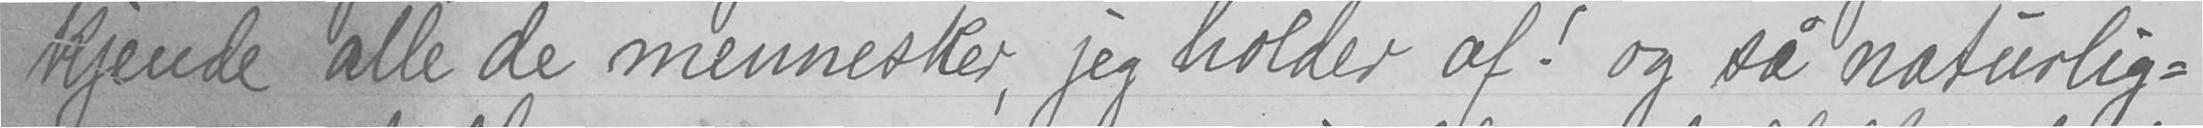kjende alle de mennesker, jeg holder af! og så naturlig-
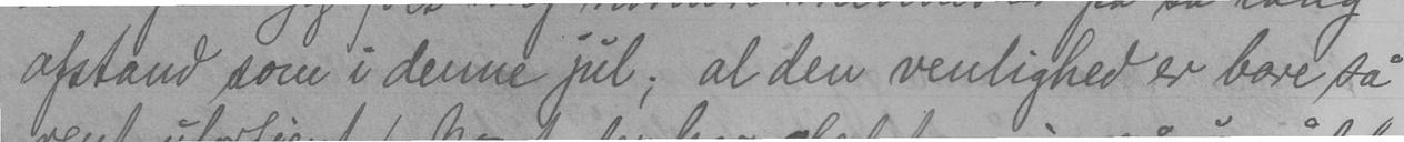afstand som i denne jul; at den venlighed er bare så
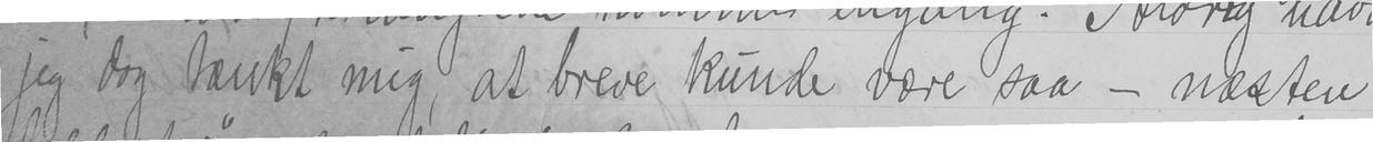jeg dog tænkt mig, at breve kunde være saa - næsten
